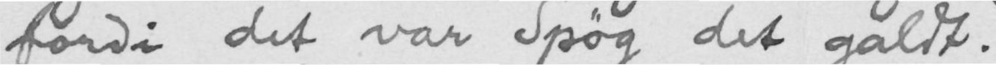fordi det var Spøg det galdt.
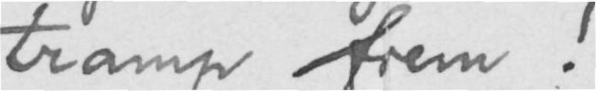tramp frem!
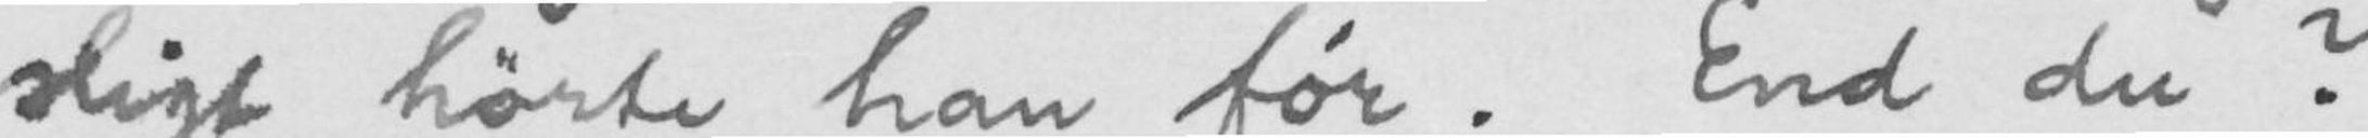sligt hørte han før. End du?
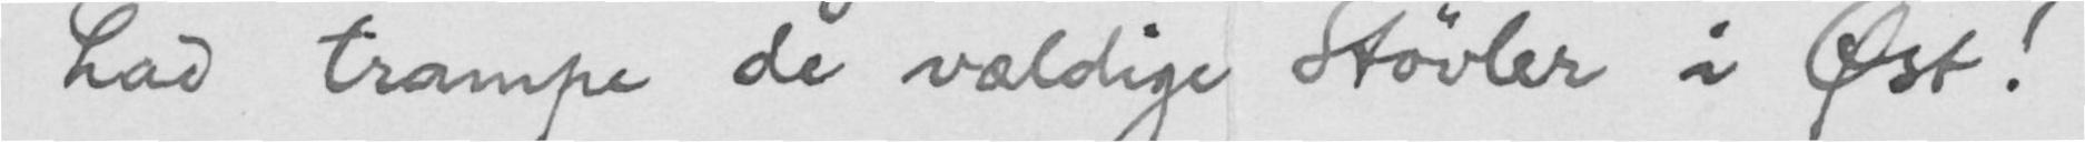Lad trampe de vældige Støvler i Øst!
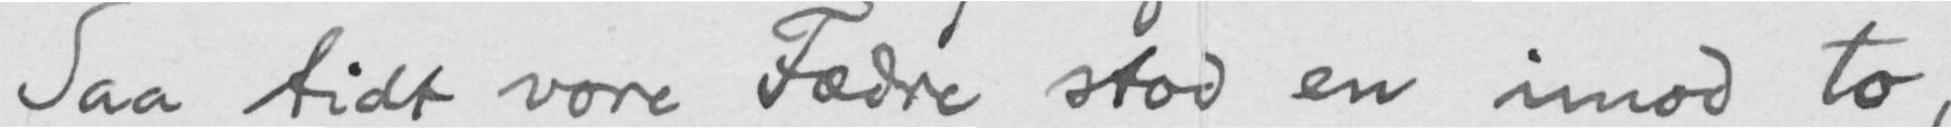Saa tidt vore Fædre stod en imod to,
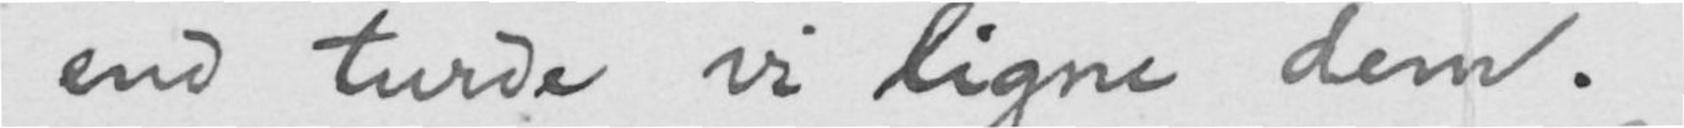end turde vi ligne dem.
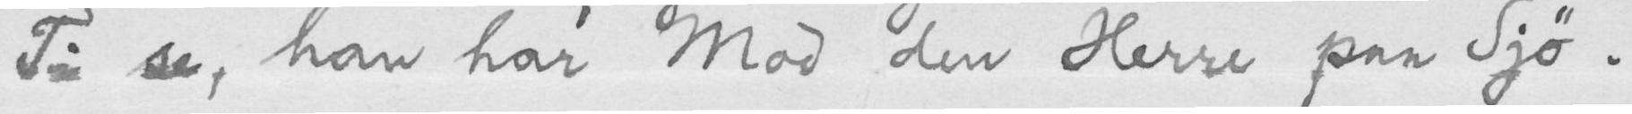Ti se, han har Mod den Herre paa Sjø.
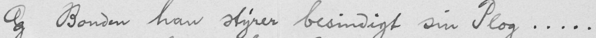Og Bonden han styrer besindigt sin Plog . . . . .
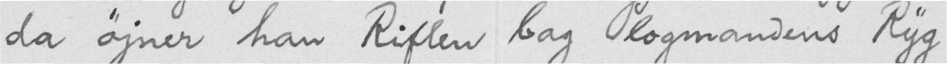da øjner han Riflen bag Plogmandens Ryg
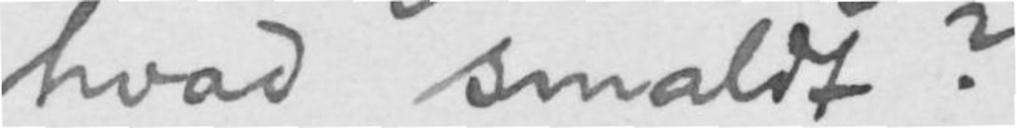hvad smaldt?
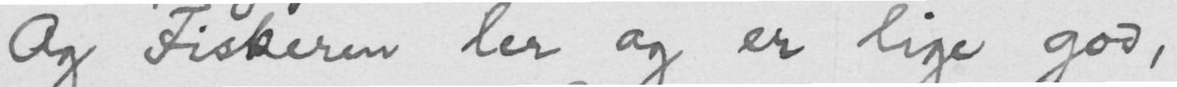Og Fiskeren ler og er lige god,
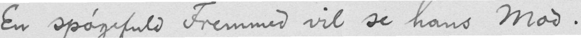En spøgefuld Fremmed vil se hans Mod.
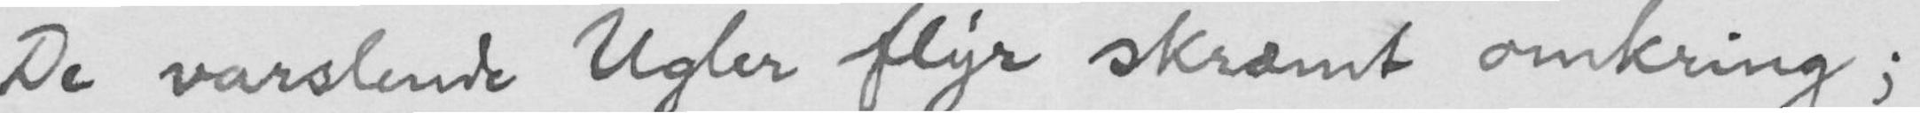De varslende Ugler flyr skræmt omkring;
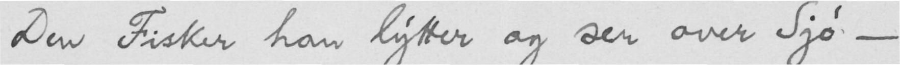Den Fisker han lytter og ser over Sjø -
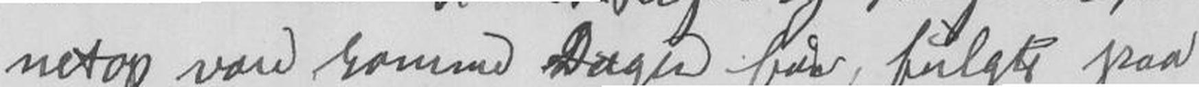netop var komme Dagen før, fulgte paa
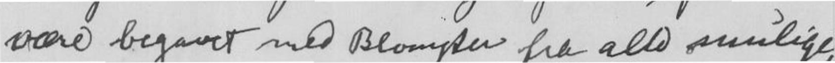være begavet med Blomster fra alle mulige,
